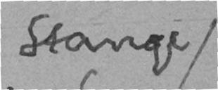Stange
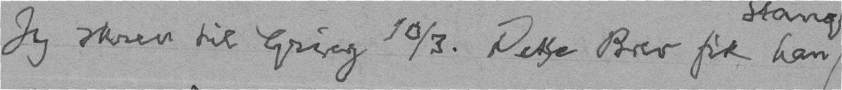Jeg skrev til Grieg 10/3. Dette Brev fik han
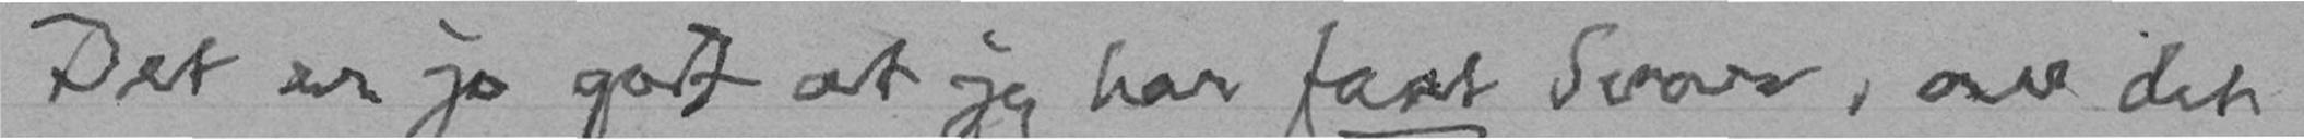Det er jo godt at jeg har faat Svar, av det
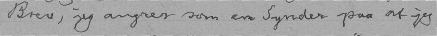Brev, jeg angrer som en Synder paa at jeg
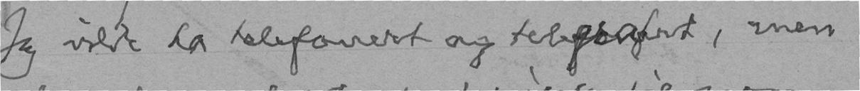Jeg vilde ha telefonert og telegrafert, men
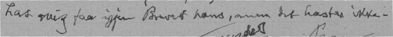Lat mig faa igjen Brevet hans, men det haster ikke.
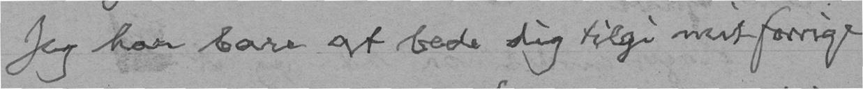Jeg har bare at bede dig tilgi mit forrige
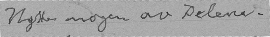Nytte nogen av Delene.
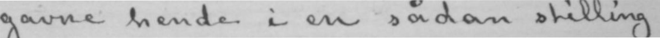gavne hende i en sådan stilling.
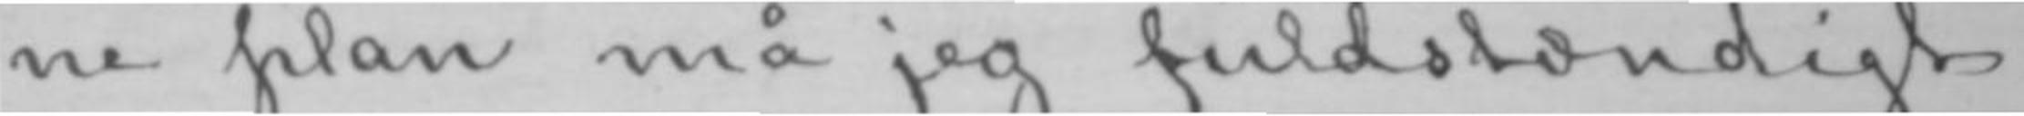ne plan må jeg fuldstændigt
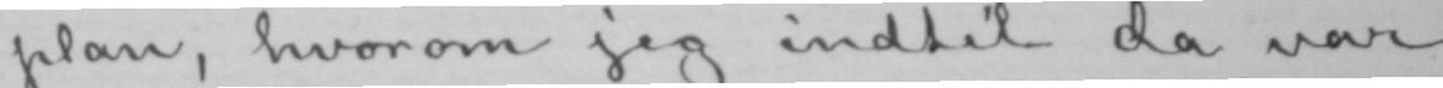plan, hvorom jeg indtil da var
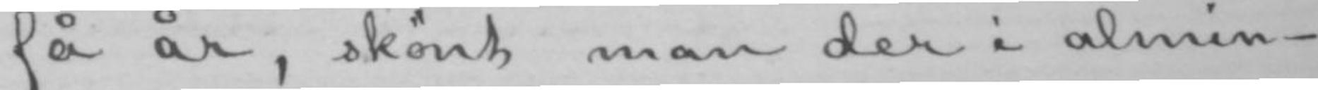få år, skønt man der i almin-
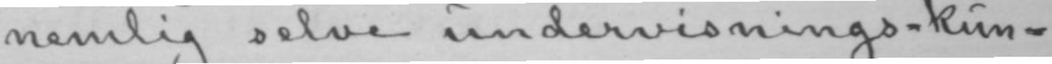nemlig selve undervisnings-kun-
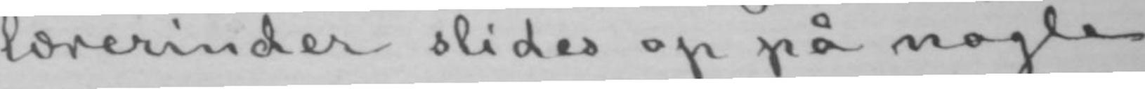lærerinder slides op på nogle
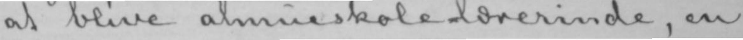at blive almueskole-lærerinde, en
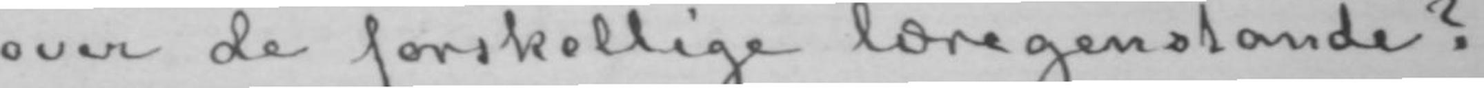over de forskellige læregenstande?
